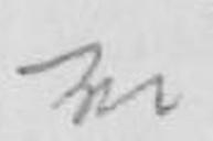vi
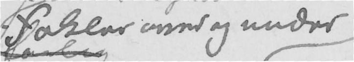Fakler over og under
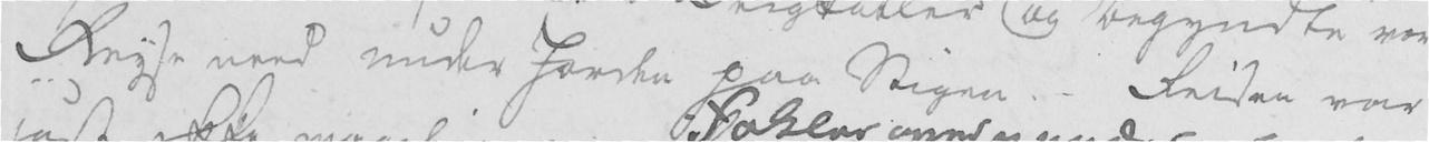Reyse need under Jorden paa Stigen. - Reisen var
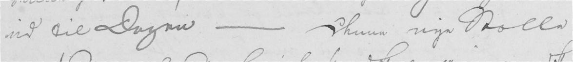ud til Dagen - Denne nye Stolle
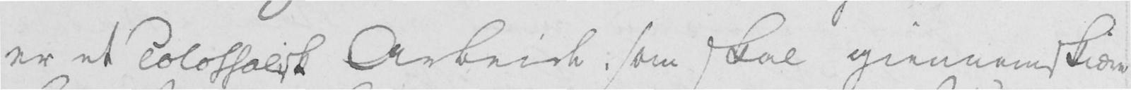er et colossalisk Arbeide, som skal giennemskiære
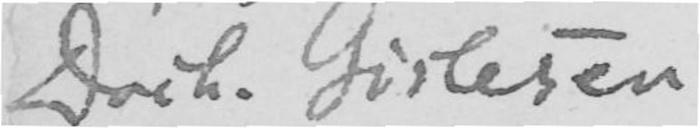Doch. Gislesen
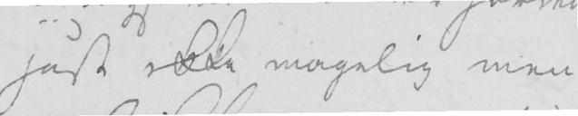just ikke magelig men
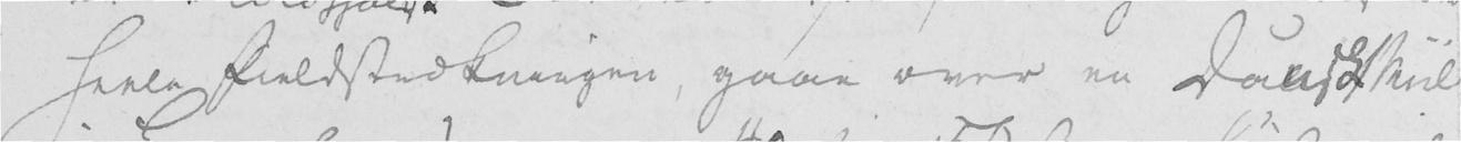heele Fieldstrækningen, gaae over en Dansk Miil
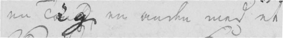en Tig en anden med et
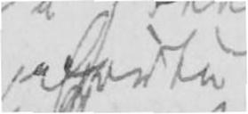iførte
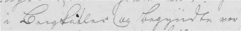i Bergkitler og begyndte vor
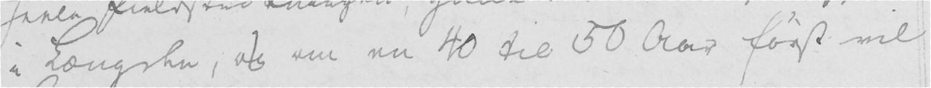i Længden, og om en 40 til 50 Aar først vil
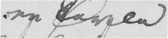en samle
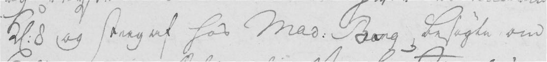Kl. 8 og steeg af hos Mad. Berg; besøgte om
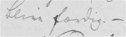blive færdig. -
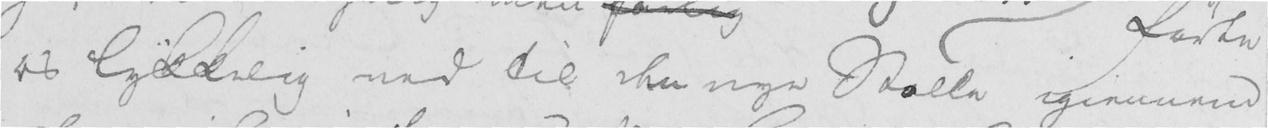os lykkelig ned til den nye Stolle igiennem
